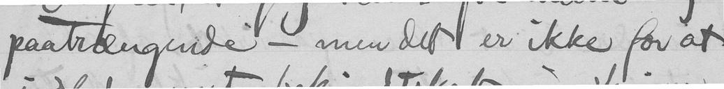paatrængende - men det er ikke for at
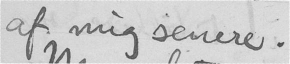af mig senere.
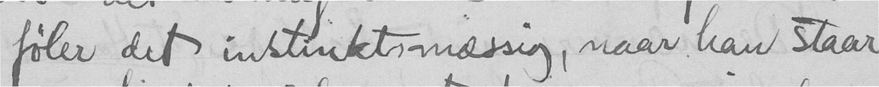føler det instinktsmæssig, naar han staar
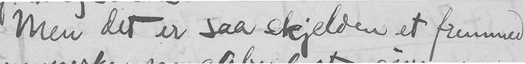Men det er saa skjelden et fremmed
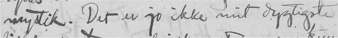mystik. Det er jo ikke mit dygtigste
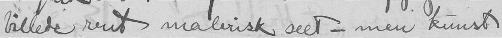billede rent malerisk seet - men kunst
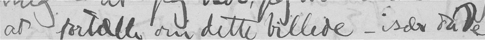at fortælle om dette billede - især da De
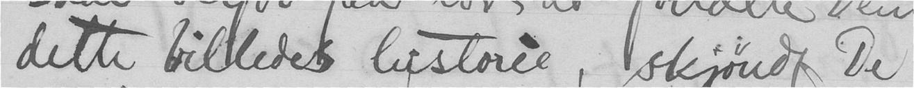dette billledes historie, skjøndt De
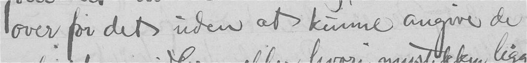over for det uden at kunne angive de
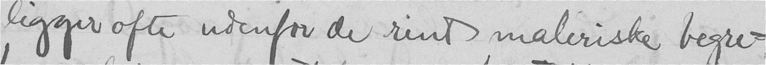ligger ofte udenfor de rent maleriske begre-
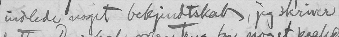indlede noget bekjendtskab, jeg skriver
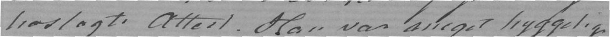hoslagte Attest. Han var meget hyggelig,
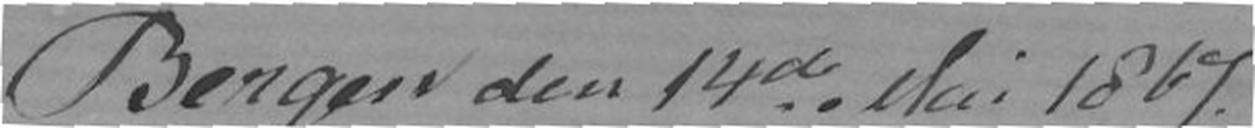Bergen den 14de Mai 1867.
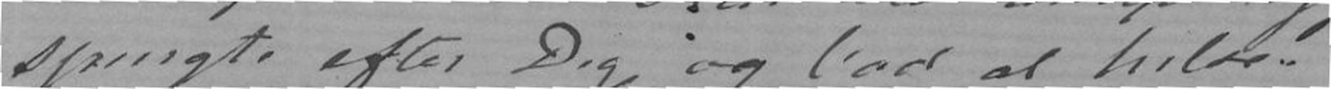spurgte efter Dig og bad at hilse.
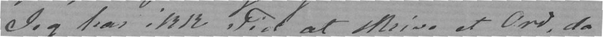Jeg har ikke Tid at skrive et Ord, da
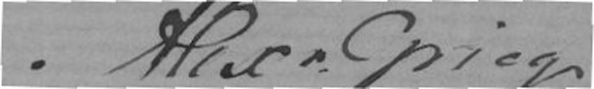Alexr. Grieg
